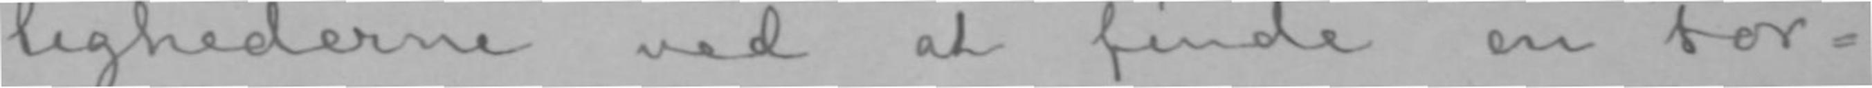lighederne ved at finde en For-
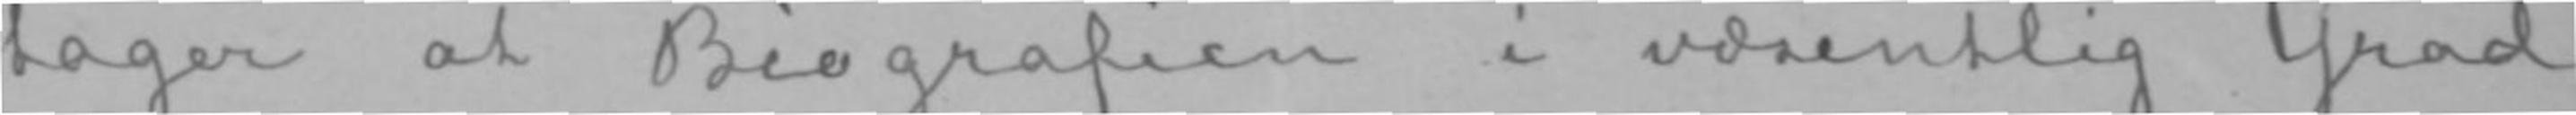tager at Biografien i væsentlig Grad
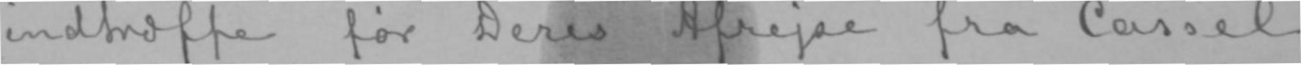indtræffe før Deres Afrejse fra Cassel
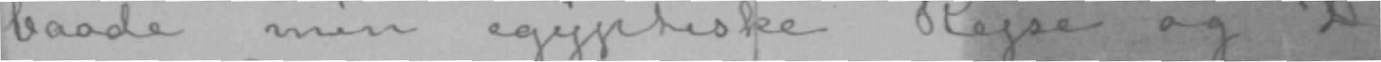baade min egyptiske Rejse og «De
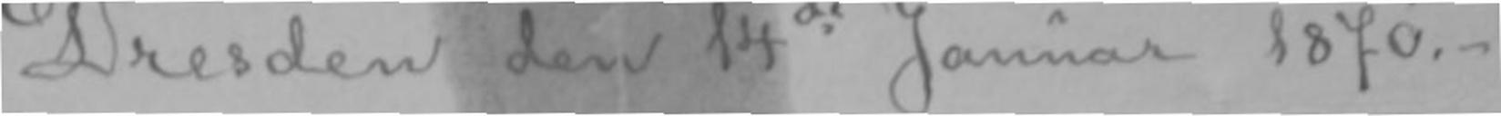Dresden den 14de Januar 1870.-
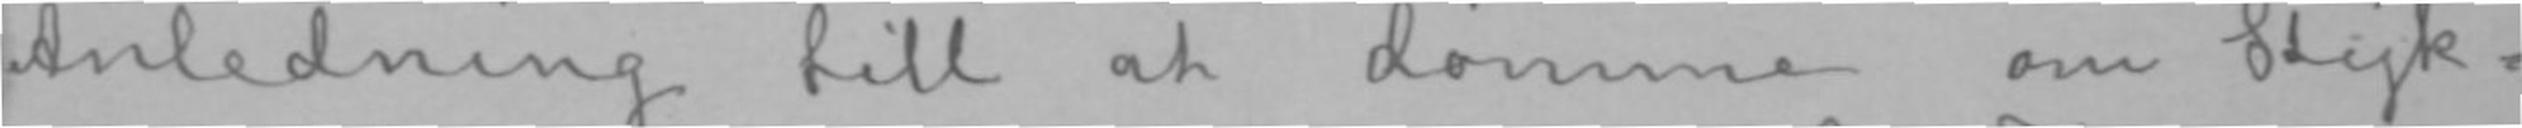Anledning till at dømme om Styk-
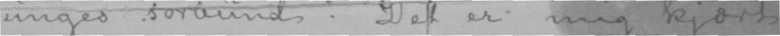unges Forbund». Det er mig kjært
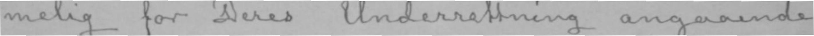melig for Deres Underrettning angaaende
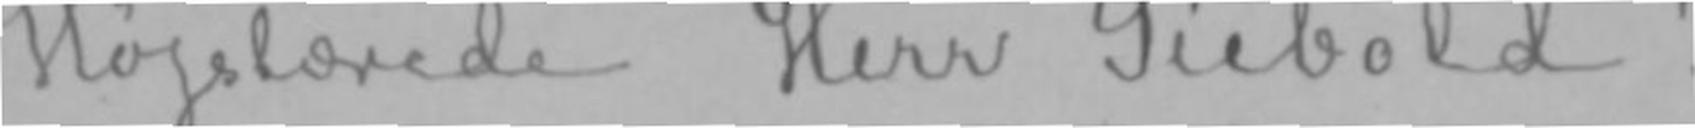Højstærede Herr Siebold!
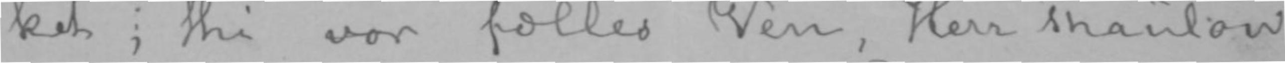ket; thi vor fælles Ven, Herr Thaulow,
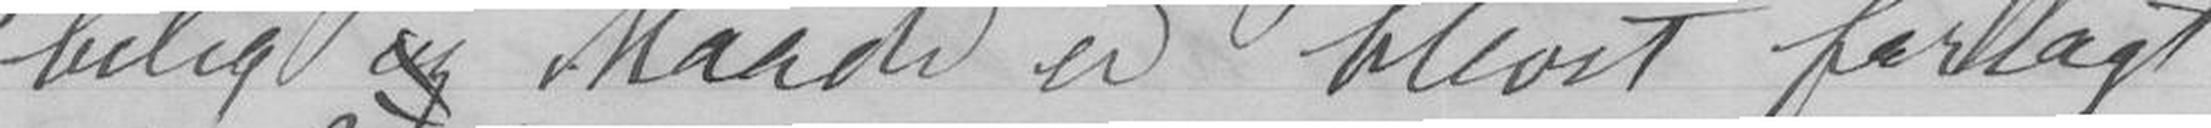belig Maade er blevet forlagt
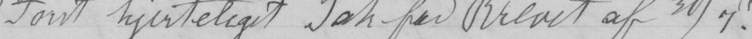Først hjerteligst Tak for Brevet af 30/7!
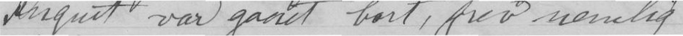August var gaaet bort, skrev nemlig
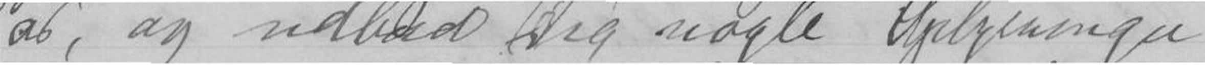os, og udbad Dig nogle Oplysninger
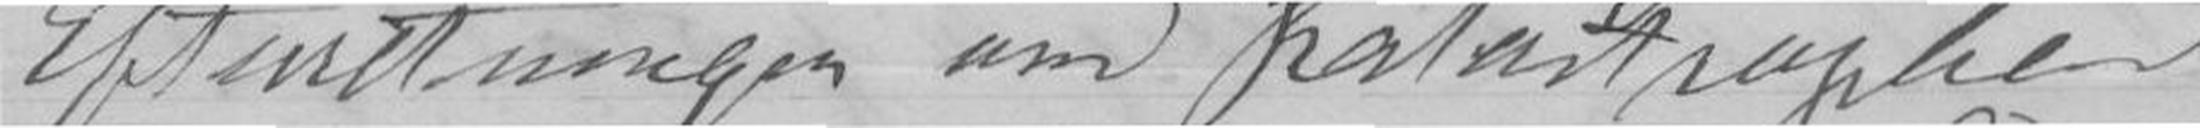Efterretningen om Katastrophen
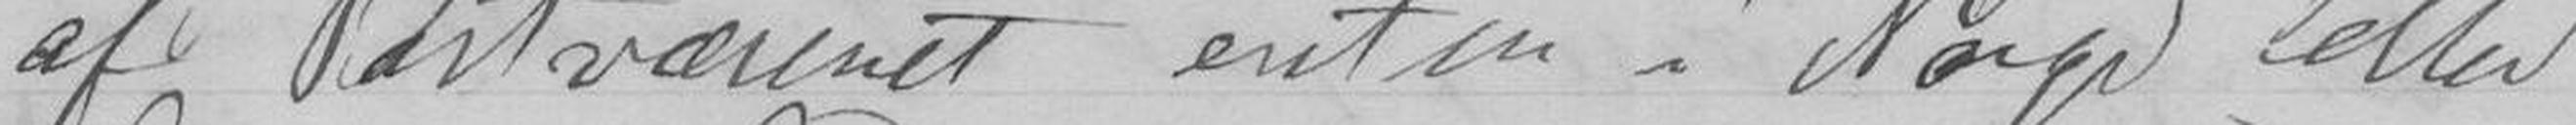af Postvæsenet enten i Norge eller
